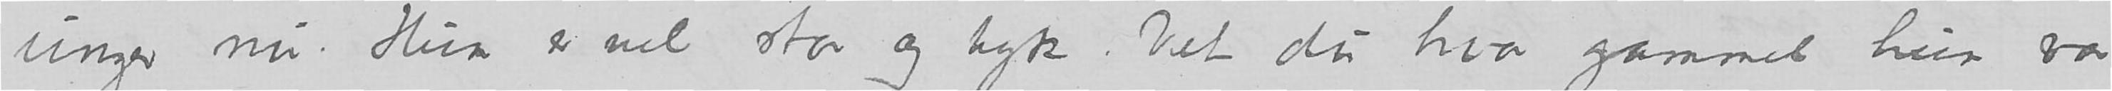unger nu. Hun er vel stor og tyk. Vet du hvor gammel hun var
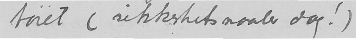tøiet (sikkerhetsnaaler dog!)
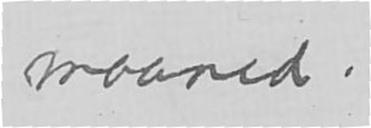maaned.
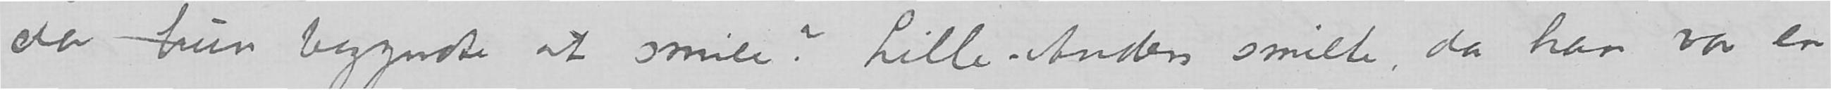da hun begyndte at smile? Lille-Anders smilte, da han var en
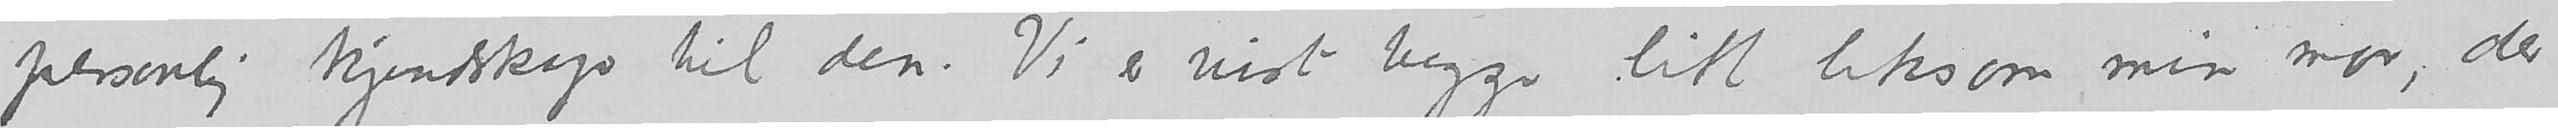personlig kjendskap til den. Vi er vist begge litt liksom min mor, der
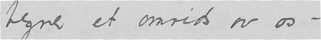tegner et omrids av os –.
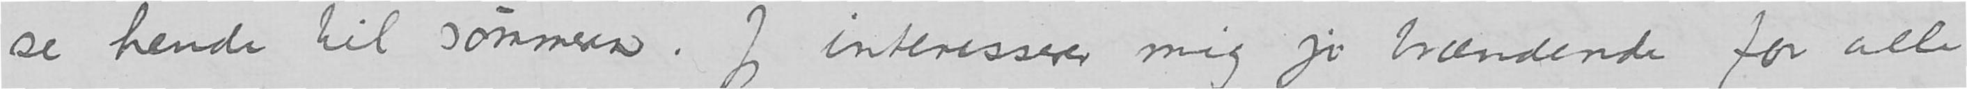se hende til sommeren. Jeg interesserer mig jo brændende for alle
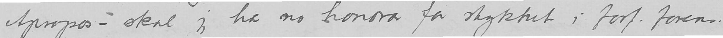Apropos – skal jeg ha no honorar for stykket i forf.forens.
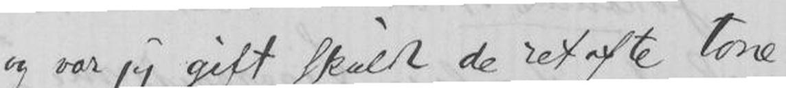og var jeg gift skulde de ret ofte tone
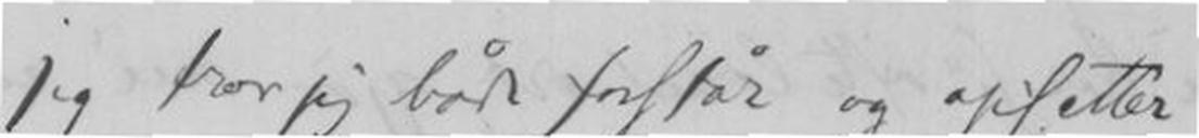jeg tror jeg både forstår og opfatter
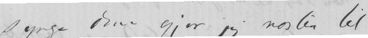synge dem gjør jeg næsten til
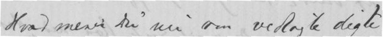Hvad mener Du nu om vedlagte digte
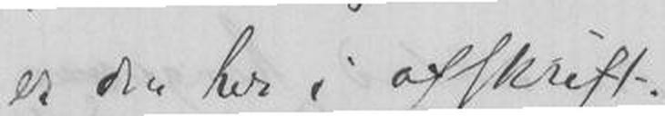er den her i afskrift.
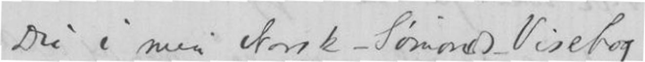Du i min Norsk-Sømondt-Visebog
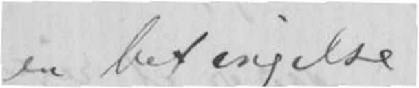en betingelse
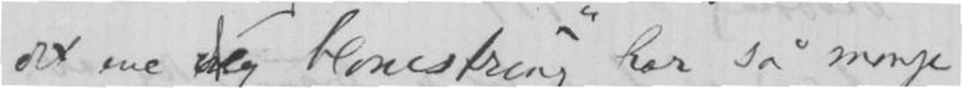det ene "Jeg blomstring" her så mange
